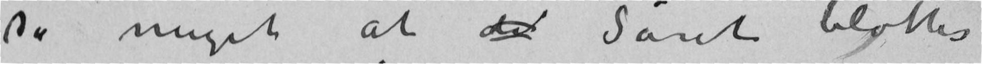så meget at det såret blottes
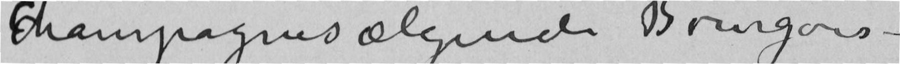Champagnesælgende Bourgois –
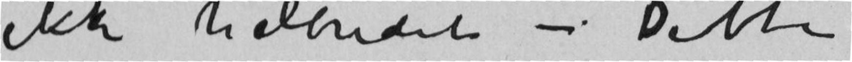ikke helbredet – Dette
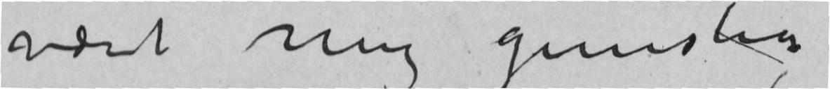været mig gunstig
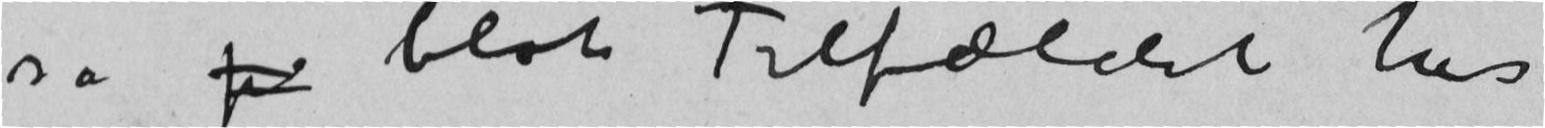så p blot Tilfældet har
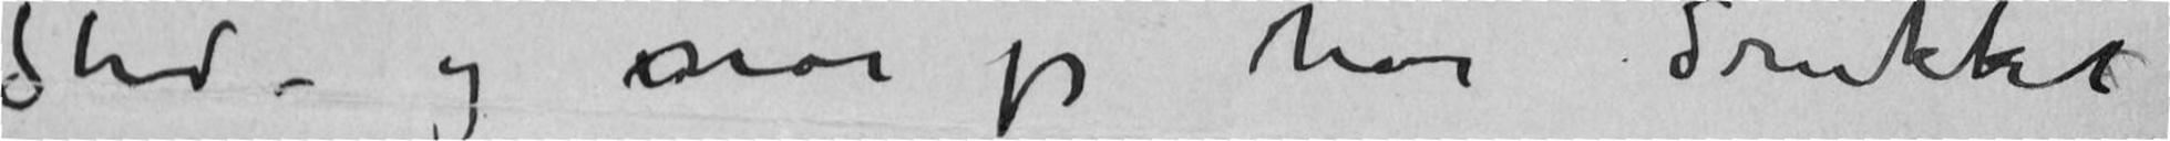Sted – og når jeg har drukket
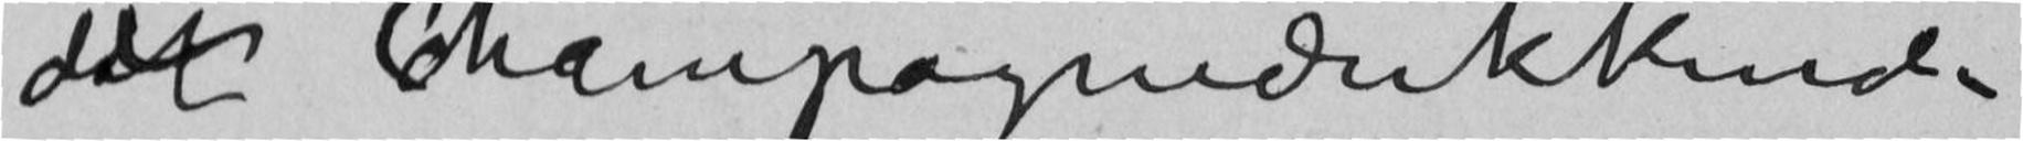dete Champagnedrikkende
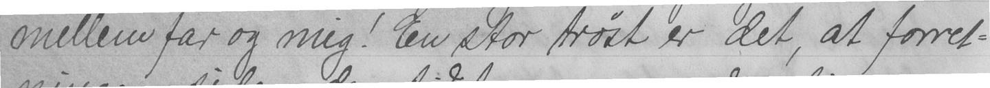mellem far og mig! En stor trøst er det, at forret-
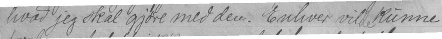hvad jeg skal gjøre med den. Enhver vilde kunne
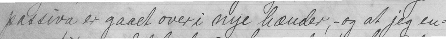passiva er gaaet over i nye hænder, - og at jeg en-
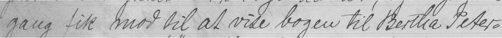gang fik mod til at vise bogen til Bertha Peter-
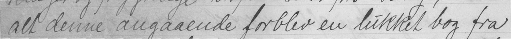alt denne angaaende forblev en lukket bog fra
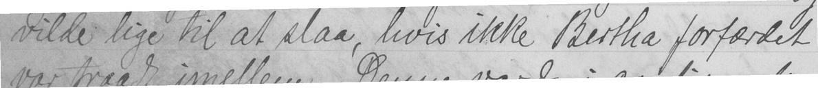vilde lige til at slaa, hvis ikke Bertha forfærdet
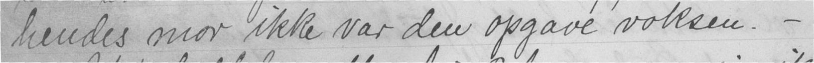hendes mor ikke var den opgave voksen. -
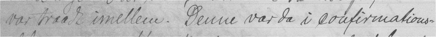var traadt imellem. Denne var da i confirmations-
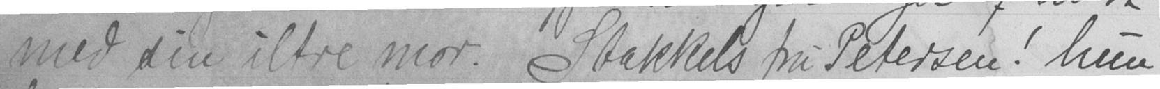med sin iltre mor. Stakkels fru Petersen! hun
